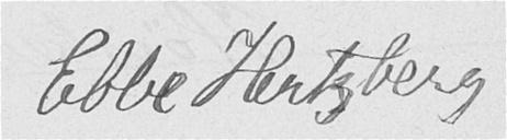Ebbe Hertzberg.
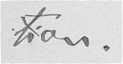tion.
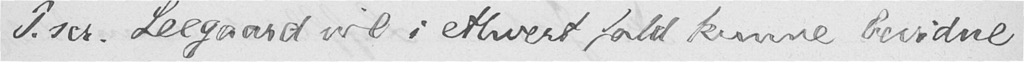P. scr. Leegaard vil i ethvert fald kunne bevidne
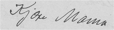Kjære Mama
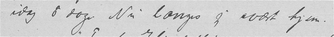idag 8 dage. Nu længes jeg svært hjem.
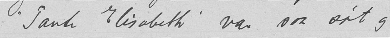Tante Elisabeth var saa søt og
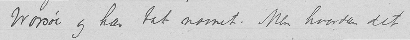Worsøe og har tat navnet. Men hvordan det
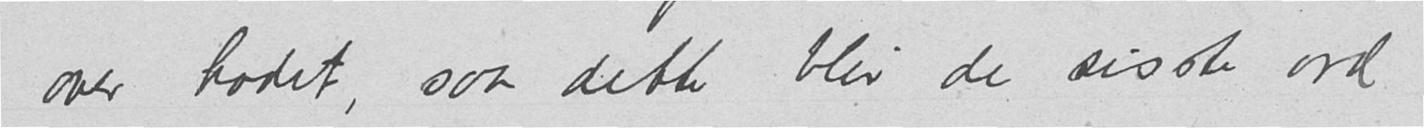over hodet, saa dette blir de sisste ord
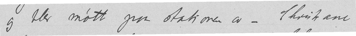og blev møtt paa stationen av – Christiane
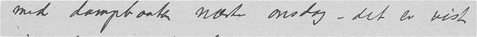med dampbaaten næste onsdag – det er vist
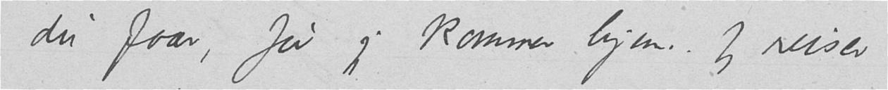du faar, før jeg kommer hjem. Jeg reiser
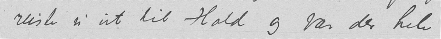reiste vi ut til Hald og var der hele
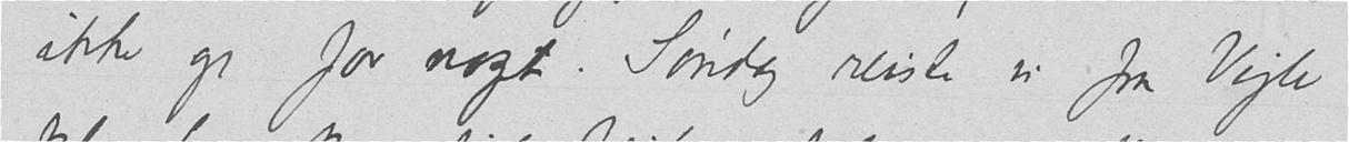ikke op for noget. Søndag reiste vi fra Vejle
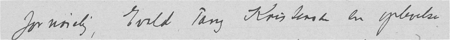fornøielig, Evald Tang Kristensen en oplevelse
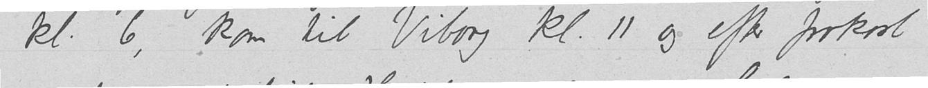kl. 6, kom til Viborg kl. 11 og efter frokost
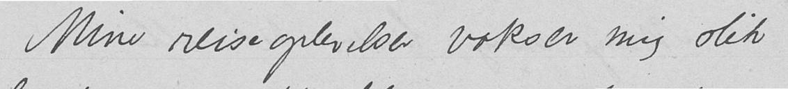Mine reiseoplevelser vokser mig slik
